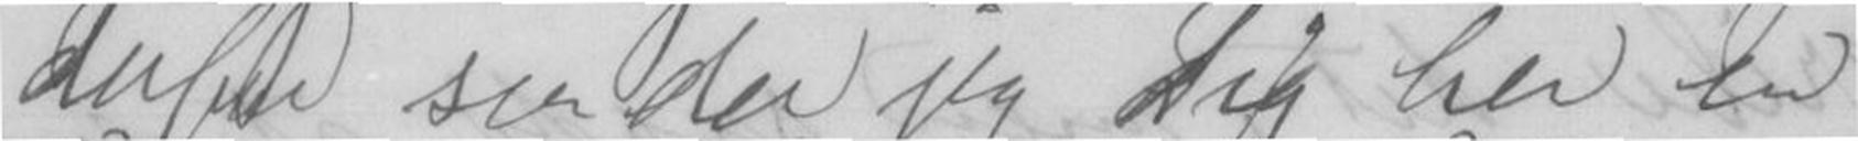derfor sender jeg Dig her en
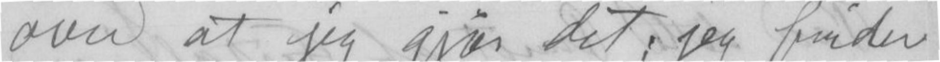over at jeg gjør det; jeg finder
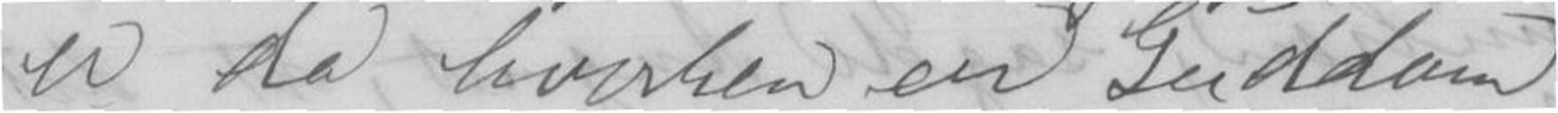er da hverken en Guddom
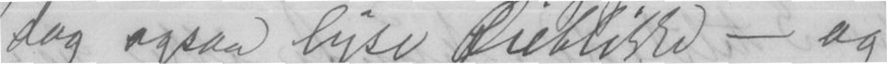dog ogsaa lyse Øieblikke - og
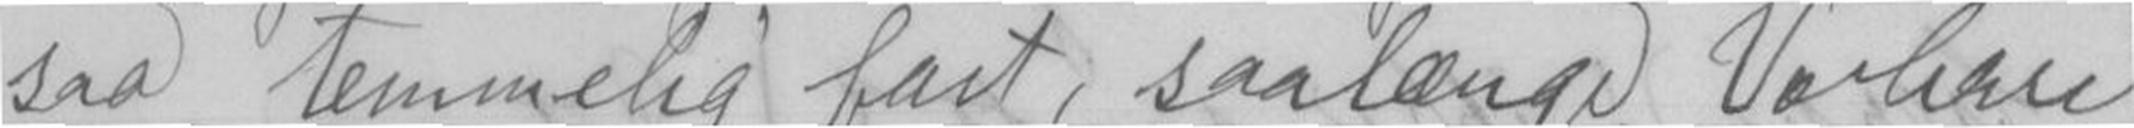saa temmelig fast, saalænge Vorherre
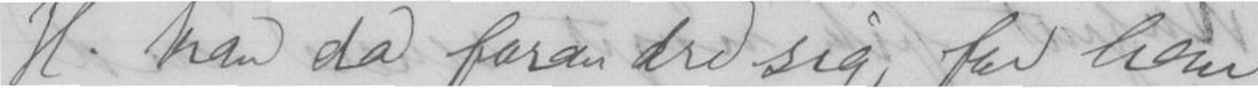H. kan da forandre sig, for han
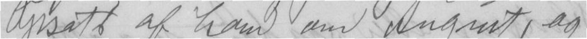Opsats af ham om August, og
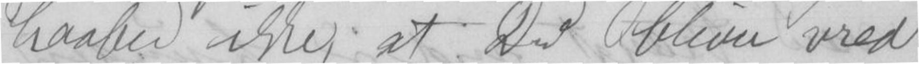haaber ikke, at Du bliver vred
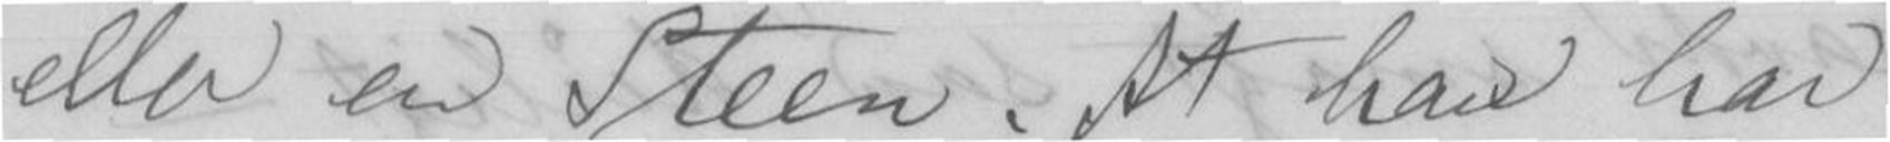eller en Steen. At han har
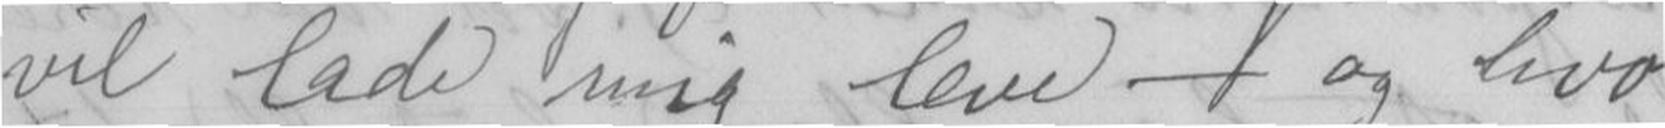vil lade mig leve - og hva
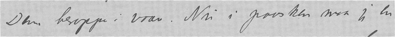Dem heroppe i vaar. Nu i paasken maa jeg en
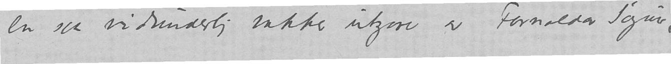en saa vidunderlig vakker utgave av Fornoldar Sögur,
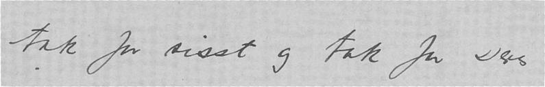tak for sisst og tak for Deres
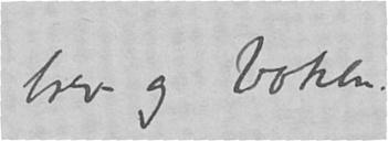brev og boken.
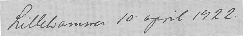Lillehammer 10. april 1922.
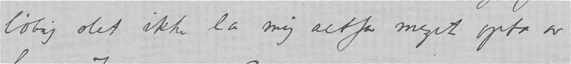løbig slet ikke la mig altfor meget opta av
